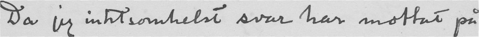Da jeg intetsomhelst svar har mottat på
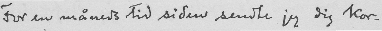For en måneds tid siden sendte jeg dig kor-
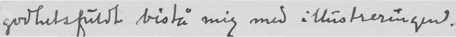godhetsfuldt bistå mig med illustreringen.
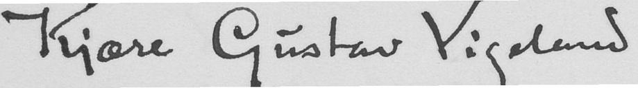Kjære Gustav Vigeland
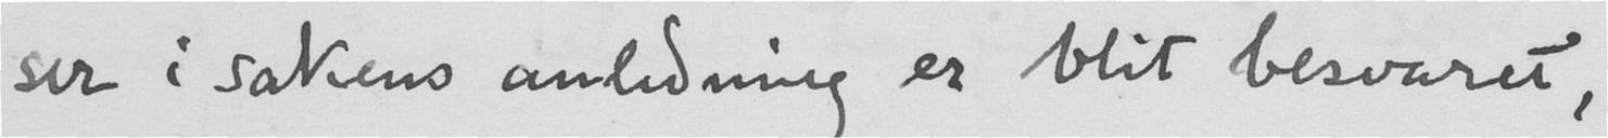ser i sakens anledning er blit besvaret,
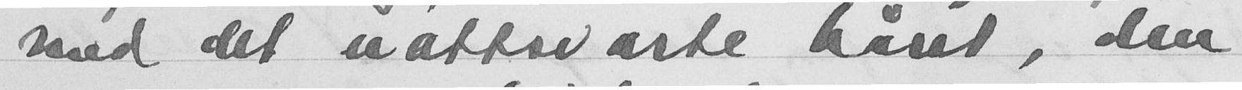med det nattsvarte bånd, den
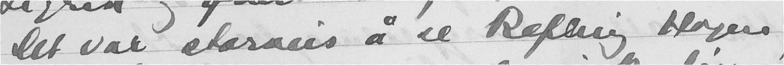Det var storveis å se Reflig Hagen
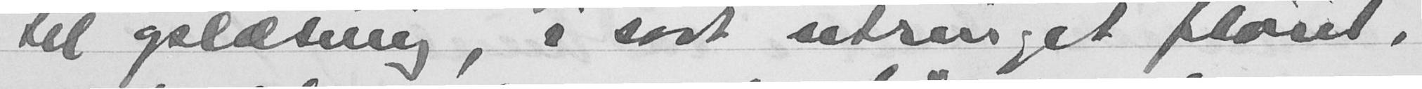til oplæring, i sort utringet flønt,
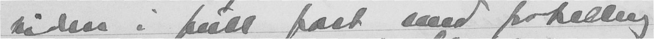tiden i full fart med fortelling
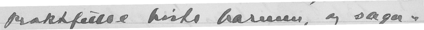praktfulle hvite barmen og saga-
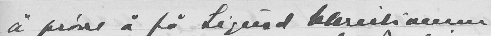å prøve å få Sigurd Christiansen
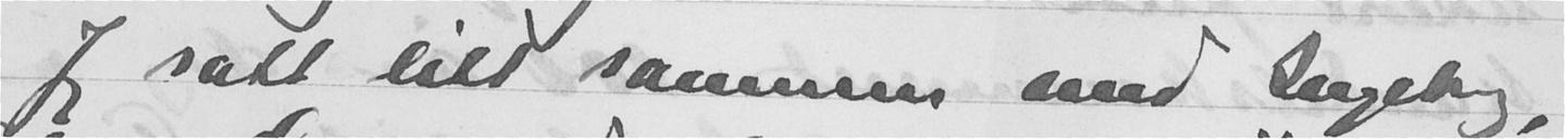Jeg satt litt sammen med Ingeborg,
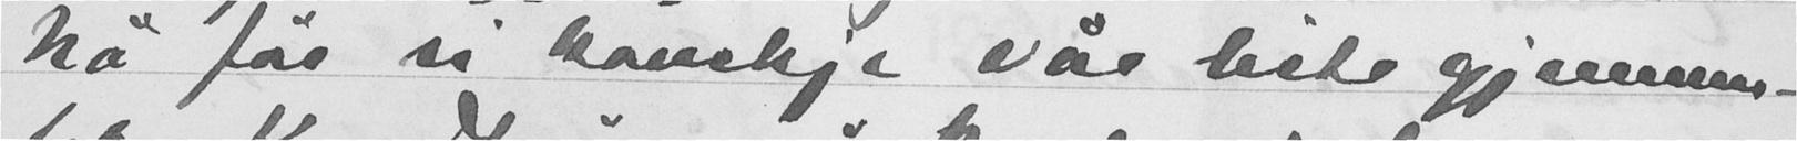Nå får vi kanskje vår liste gjennom.
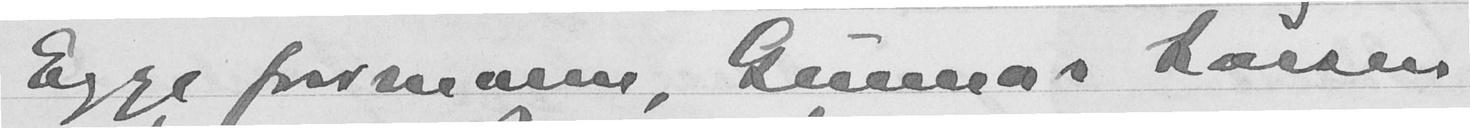Egge formann, Gunnar Larsen
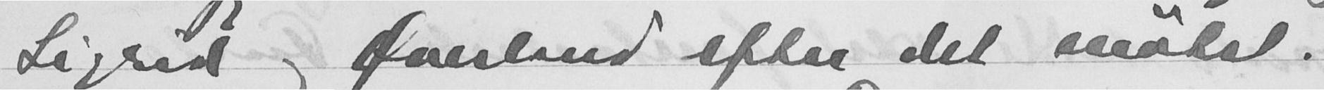Sigrid og Øverland efter det møtet.
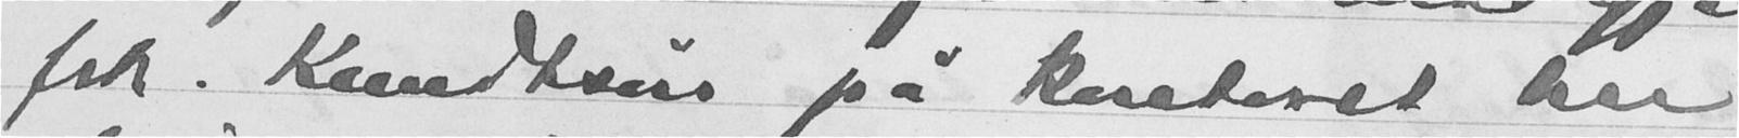frk. Kundteus på kontoret her
